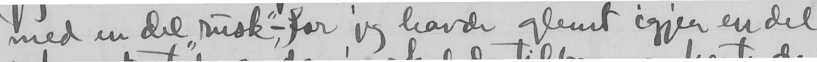med en del "rusk" - for jeg havde glemt igjen en del
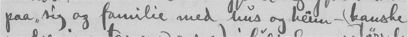paa "sig og familie med hus og heim - (kanske
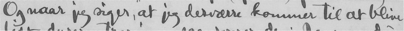Og naar jeg diger, at jeg desværre kommer til at blive
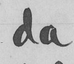da
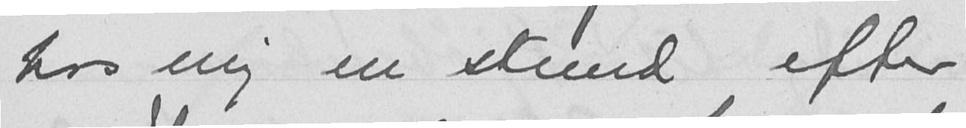hos mig en stund efter
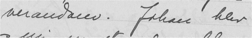verandaen. Johan blev
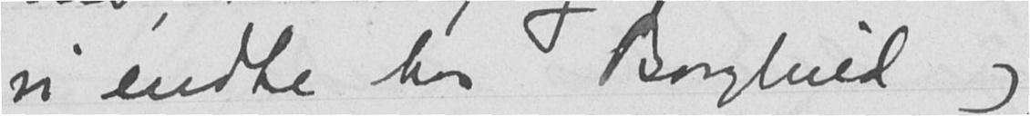vi endte hos Borghild og
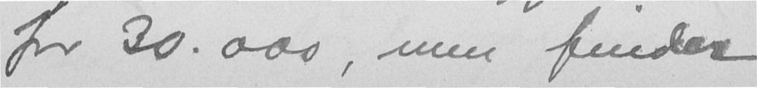for 30.000, men finder
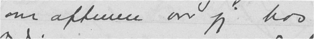om aftenen var jeg hos
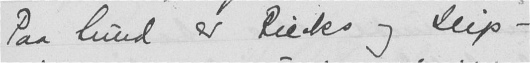Paa Sund er Riecks og Seip -
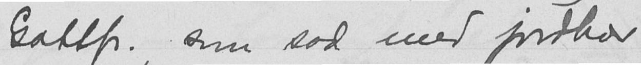Gottfr. som sad med jordbær
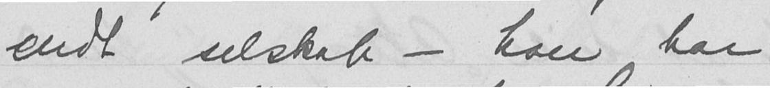endt selskab - han har
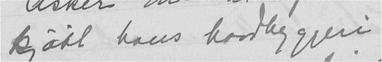kjøbt hans baadbyggeri
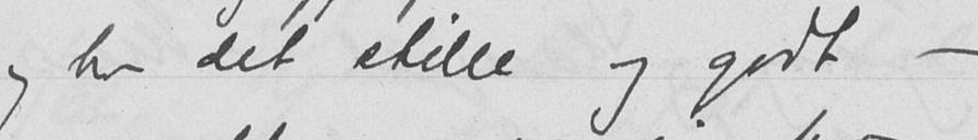og har det stille og godt -
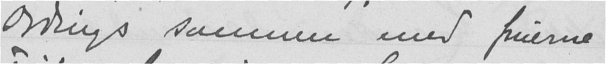Ordings sammen med fruerne
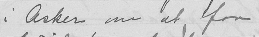i Asker om at faa
